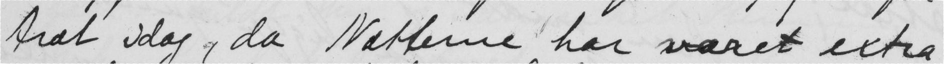træt idag, da Nætterne har været extra
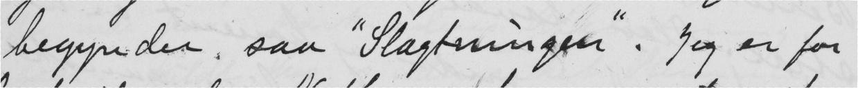begynder sau "Slagtningen". Jeg er for
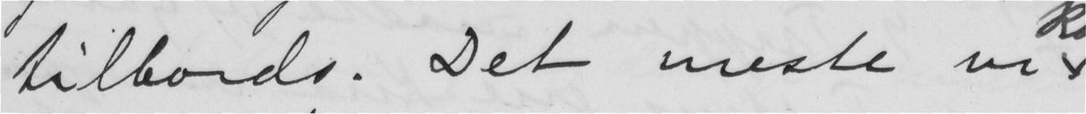tilbords. Det meste vi
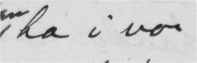ha i vor
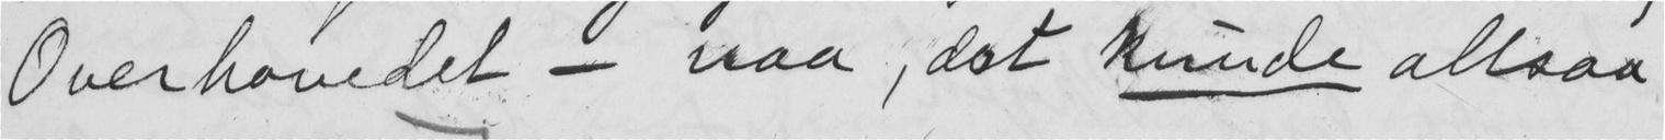Overhovedet - naa, det kunde altsaa
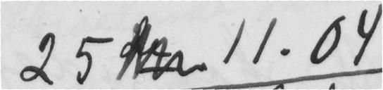25 11.04
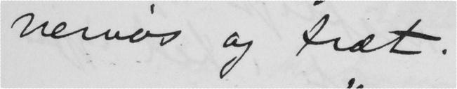nervøs og træt.
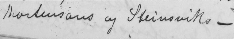Mortensons og Steinsviks -
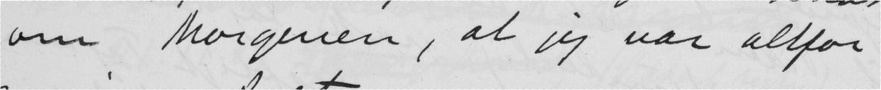om Morgenen, at jeg var altfor
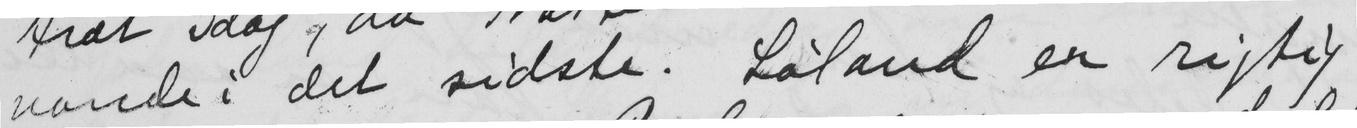vonde i det sidste. Løland er rigtig
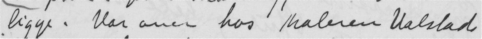ligge. Var over hos Maleren Valstad
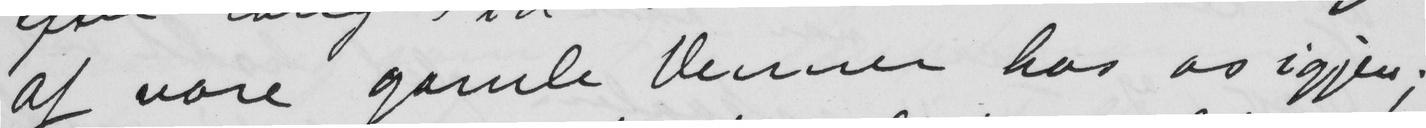af vore gamle Venner hos os igjen;
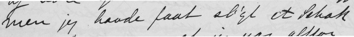men jeg havde faat sligt et Schok
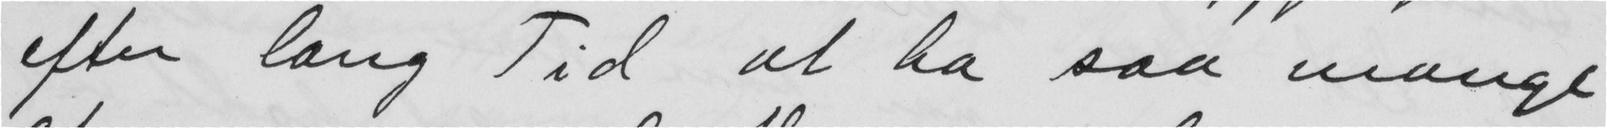efter lang Tid at ha saa mange
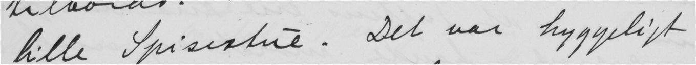lille Spisestue. Det var hyggeligt
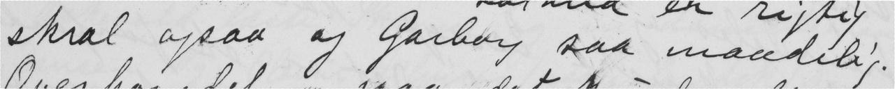skral ogsaa og Garborg saa maadelig.
